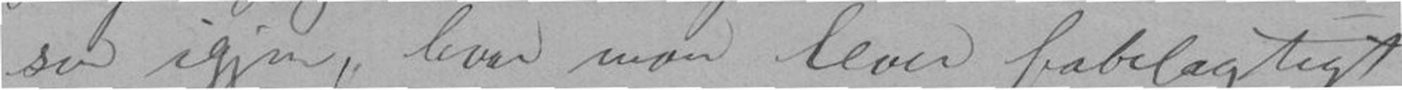sen igjen, hvor man lever fabelagtigt
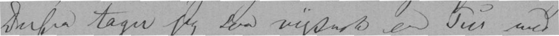Derfra tager jeg saa vistnok en Tur med
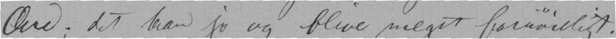Ære; det kan jo og blive meget fornøieligt.
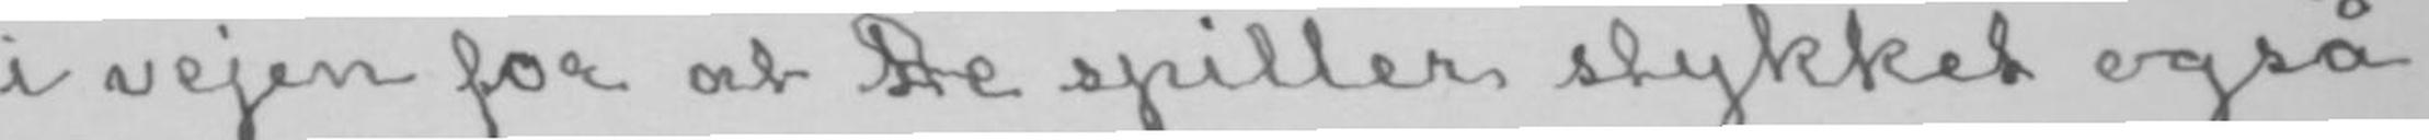i vejen for at De spiller stykket også
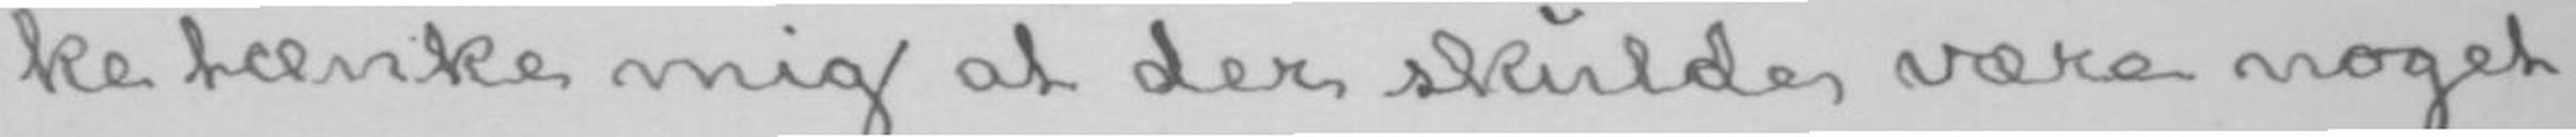ke tænke mig at der skulde være noget
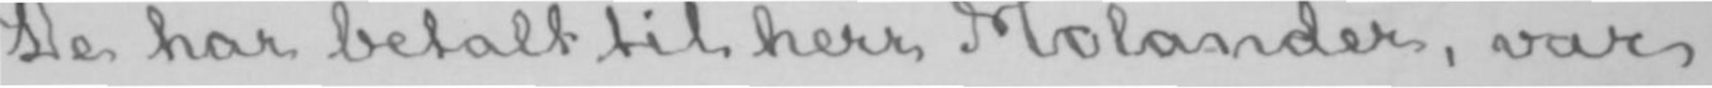De har betalt til herr Molander, var
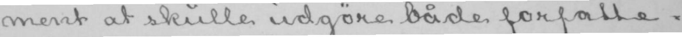ment at skulle udgøre både forfatte-
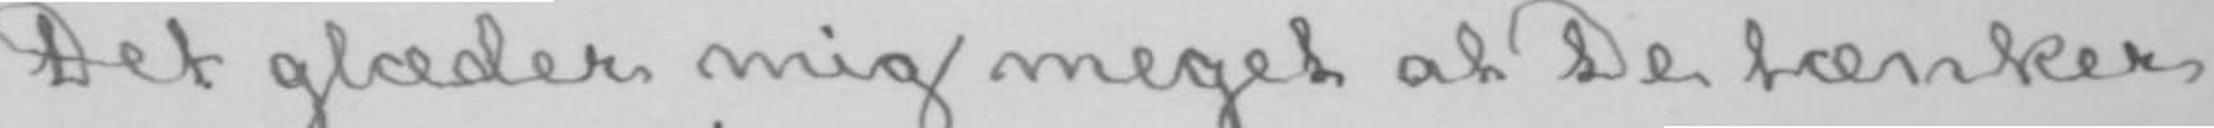Det glæder mig meget at De tænker
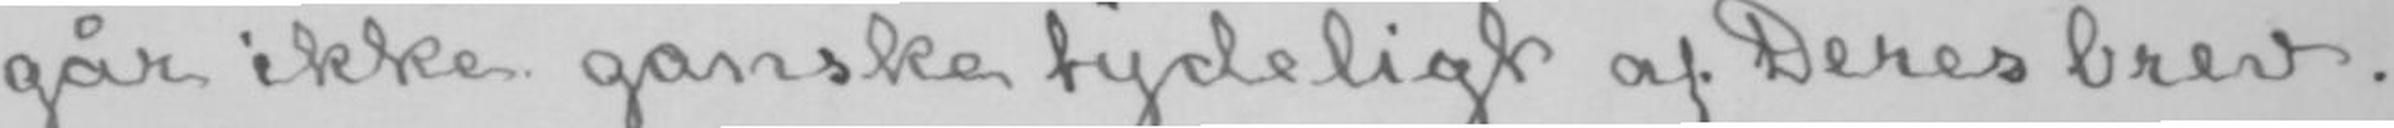går ikke ganske tydeligt af Deres brev.
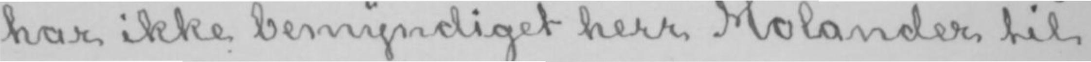har ikke bemyndiget herr Molander til
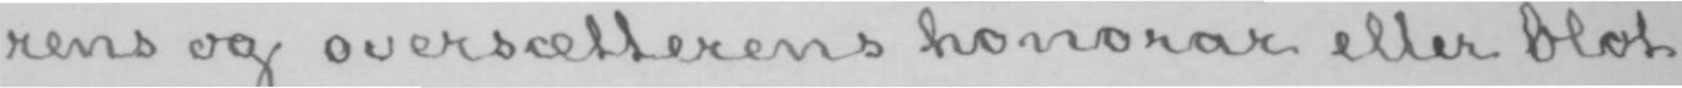rens og oversætterens honorar eller blot
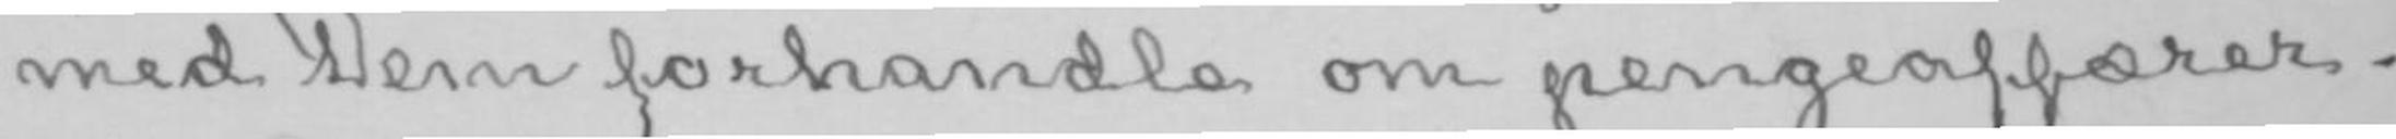med Dem forhandle om pengeaffærer.
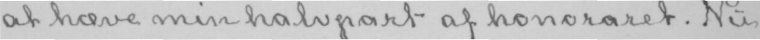at hæve min halvpart af honoraret. Nu
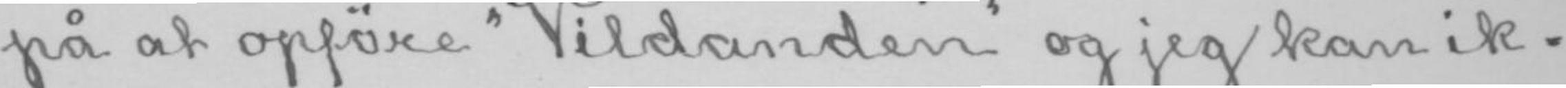på at opføre «Vildanden» og jeg kan ik-
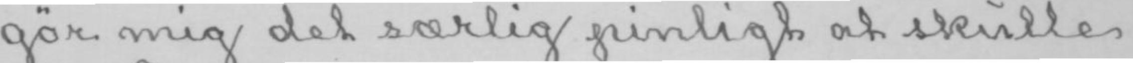gør mig det særlig pinligt at skulle
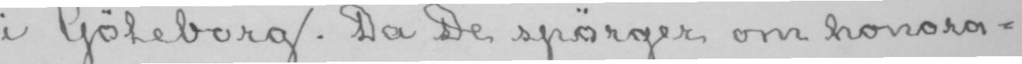i Göteborg. Da De spørger om honora-
